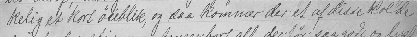kelig et kort øieblik, og saa kommer der et af disse kolde
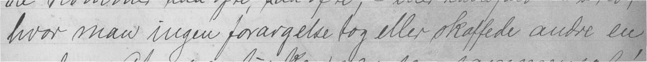hvor man ingen forargelse tog eller skaffede andre en
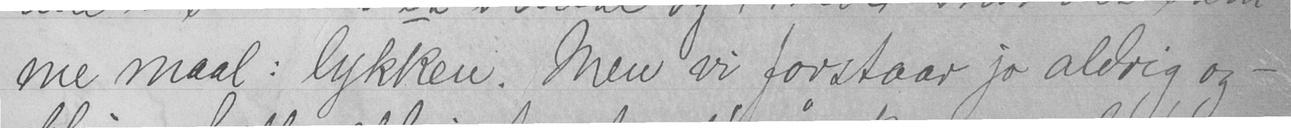me maal: lykken. Men vi forstaar jo aldrig og -
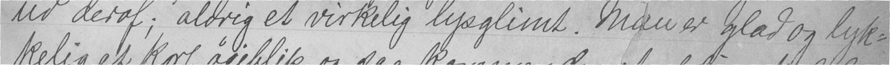ud deraf; aldrig et virkelig lysglimt. Man er glad og lyk-
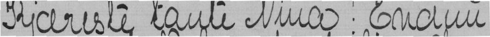Kjæreste tante Nina! Endnu
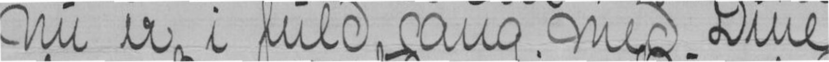nu er i fuld gang med Dine
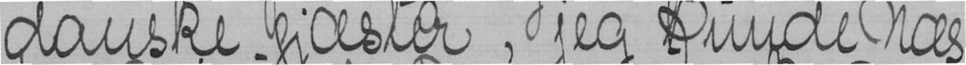danske gjæster, jeg kunde næs
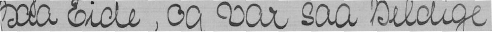paa Eide, og var saa heldige
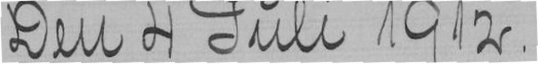Den 4 Juli 1912.
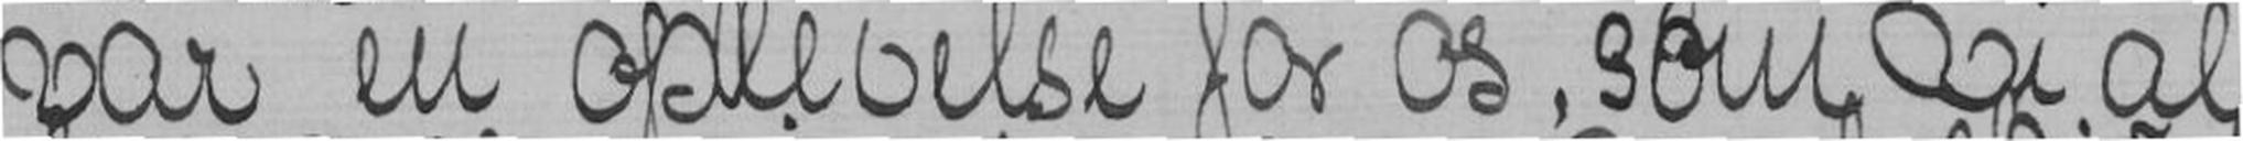var en oplevelse for os, som vi al
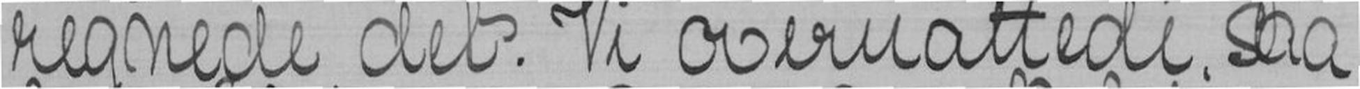regnede det. Vi overnattede saa
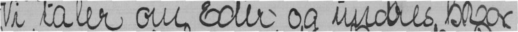Vi taler om Eder og undres hvor
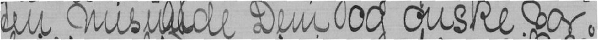ten misunde Dem og ønske vor
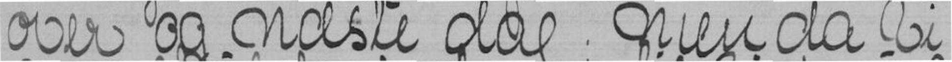over og næste dag. Men da vi
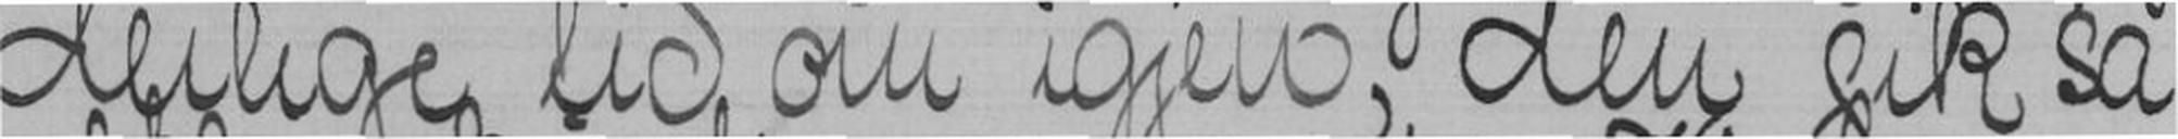deilige tid om igjen, den gik så
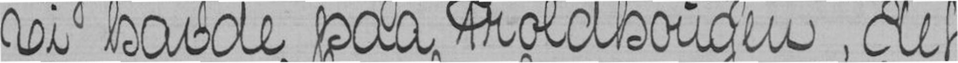vi havde paa Troldhougen, det
